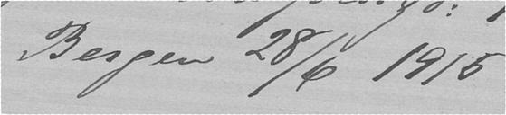Bergen 28/6 1915.
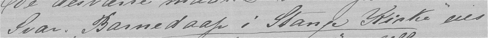Svar. "Barnedaap i Stange Kirke" eies
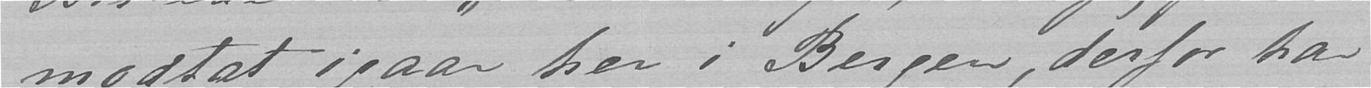modtat igaar her i Bergen, derfor har
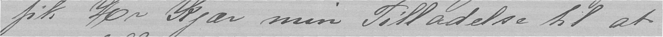fik Hr Kjær min Tilladelse til at
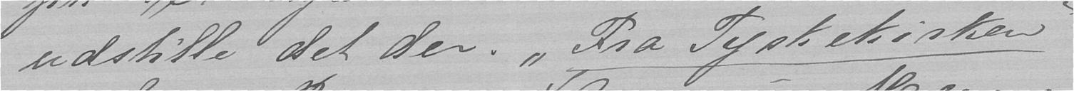udstille det der. "Fra Tyskekirken"
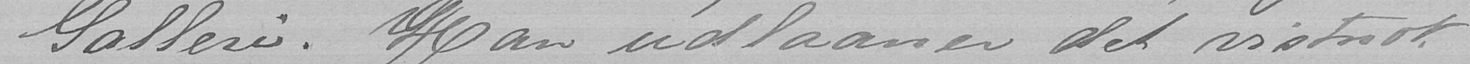Galleri. Han udlaaner det vistnok
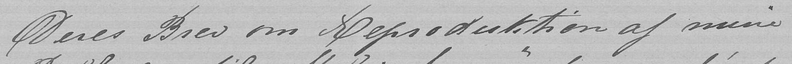Deres Brev om Reproduktion af mine
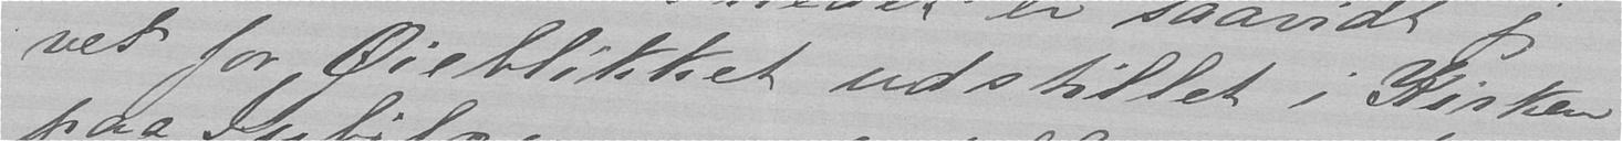vet for Øieblikket udstillet i Kirken
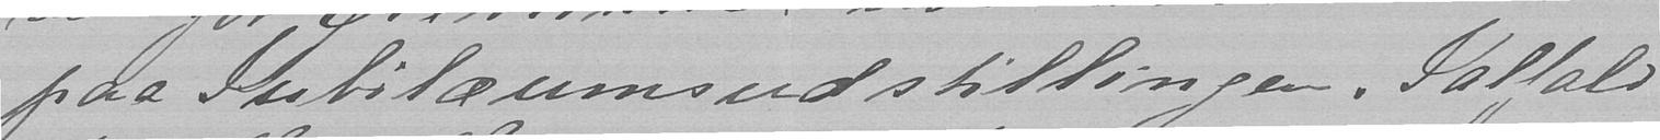paa Jubilæumsudstillingen. Ialfald
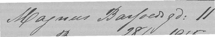Magnus Barfoedsgd. 11
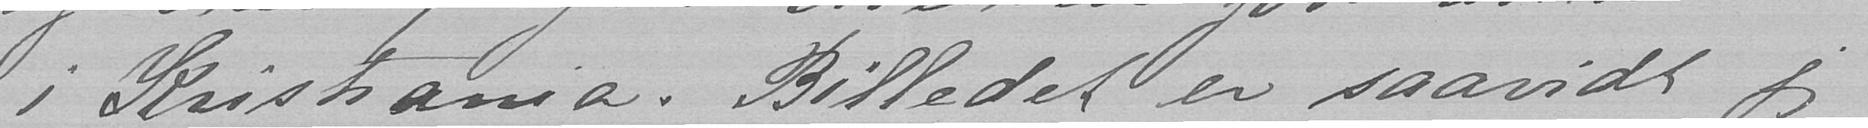i Kristiania. Billedet er saavidt jeg
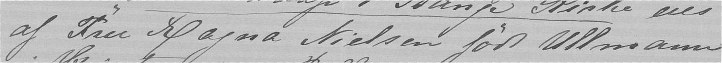af Fru Ragna Nielsen født Ullmann
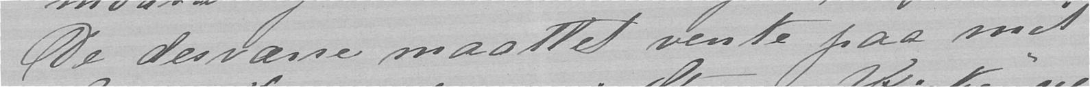De desværre maattet vente paa mit
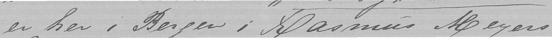er her i Bergen i Rasmus Meyers
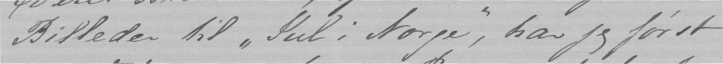Billeder til "Jul i Norge", har jeg først
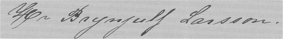Hr Brynjulf Larsson.
